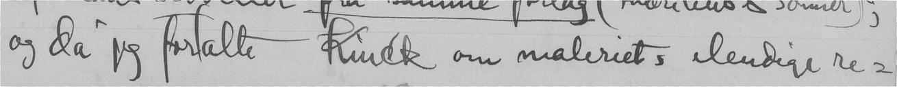og da jeg fortalte Kinck om maleriet, elendige re-
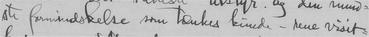ste formindskelse som tænkes kunde - rene viset-
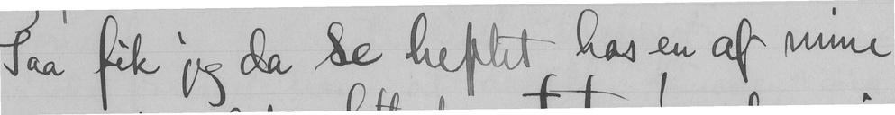Saa fik jeg da se heftet hos en af mine
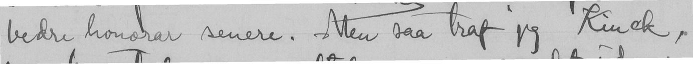bedre honorar senere. Men saa traf jeg Kinck,
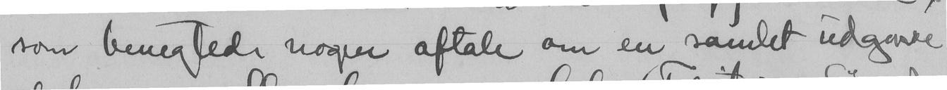som bengtede nogen aftale om en samlet udgave
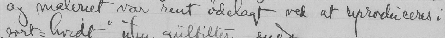og maleriet var rent ødelagt ved at reproduceres i
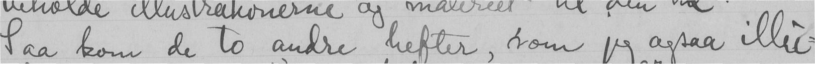Saa kom de to andre hefter, som jeg ogsaa illu-
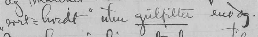"sort-hvidt" uten gulfilter endog.
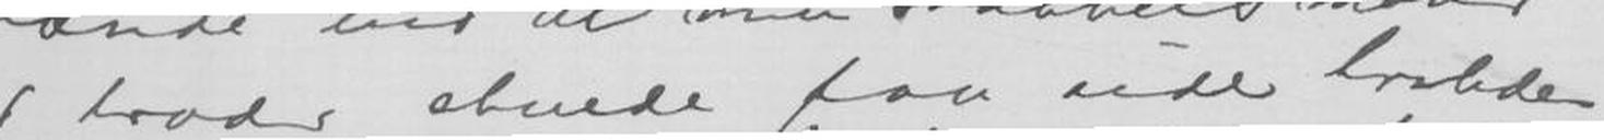og brioder skulde faa vide hvorledes
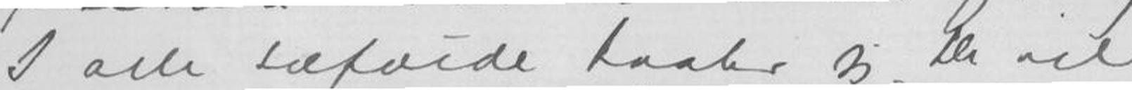I alle tilfælde haaber jeg De vil
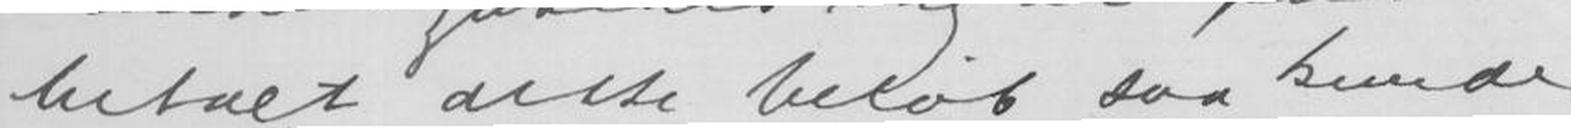betalt dette beløb saa kunde
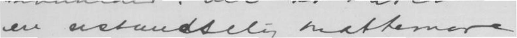en ustandselig nattemare
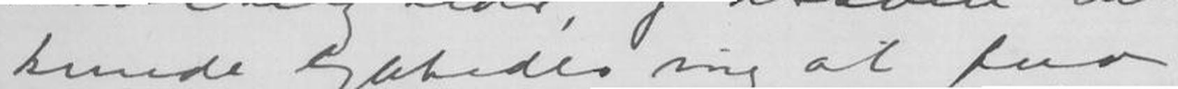kunde lykkedes mig at faa
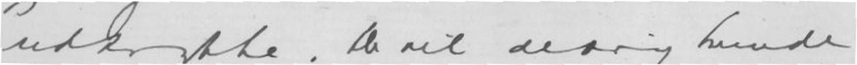udtrykke. De vil aldrig kunde
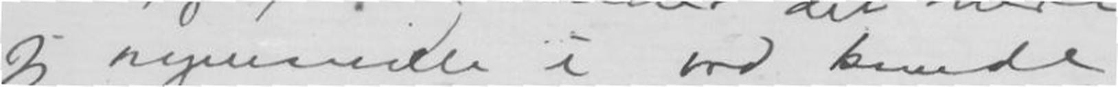jeg nogensinde i ord kunde
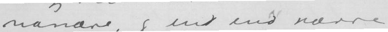vanære, og en end værre
